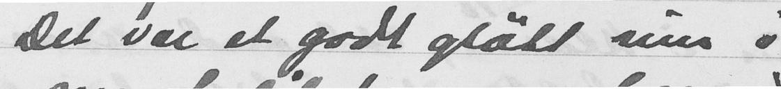Det var et godt gløtt inn i
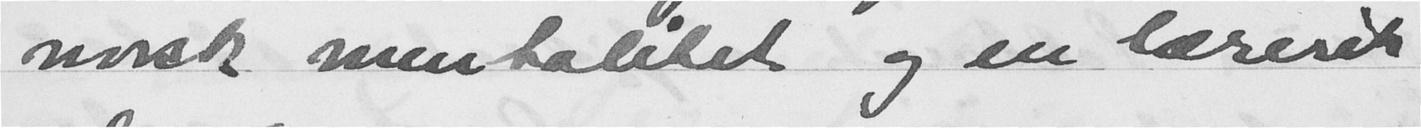norsk mentalitet og en lærerik
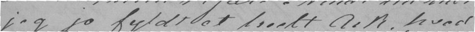jeg jo fyldt et heelt Ark, hvad
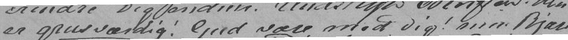er grusværdig! Gud være med Dig! min kjære
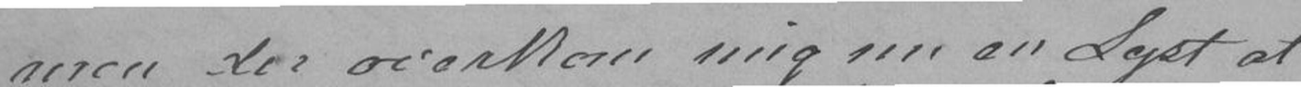men der overkom mig nu en Lyst at
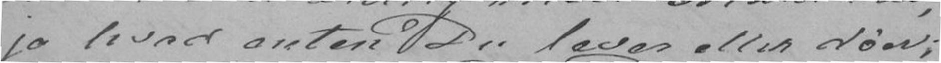jo hvad enten Du lever aller døer;
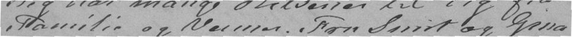Familie og Venner. Fru Smit og Gina
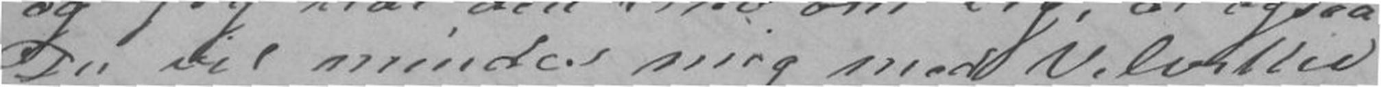Du vil mindes mig med Velvillie
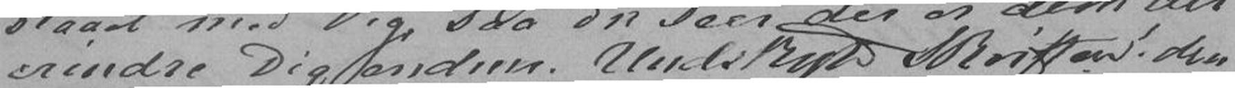erindre Dig endnu. Undskyld Skriften! den
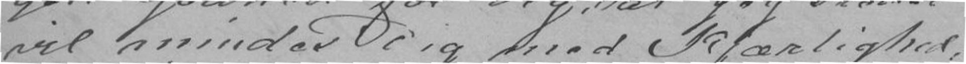vil mindes Dig med Kjærlighed,
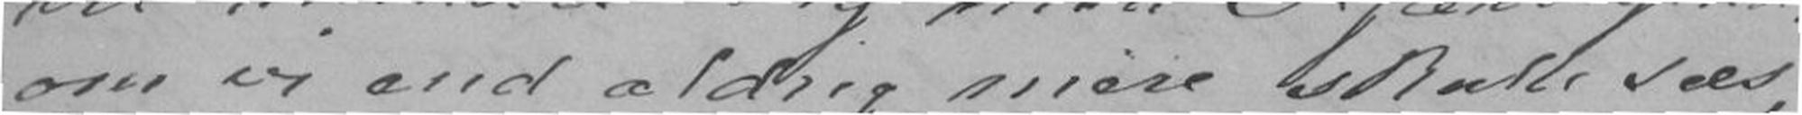om vi end aldrig mere skulle sees,
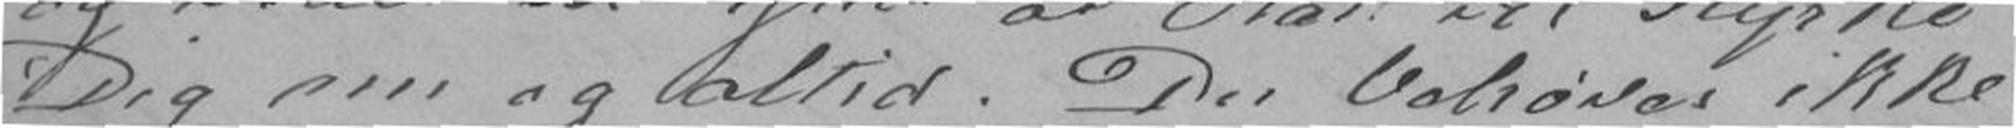Dig nu og altid. Du behøves ikke
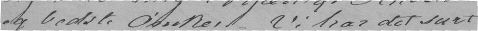og bedste Ønsker - Vi har det surt
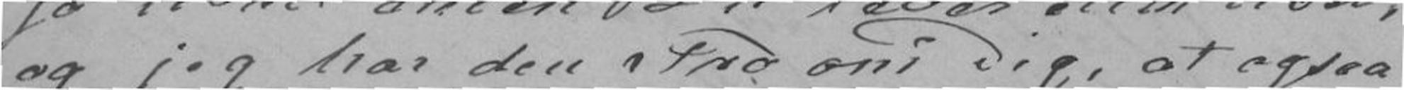og jeg har den Tro om Dig, at ogsaa
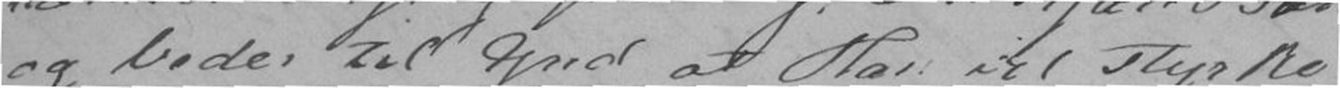og beder til Gud at Han vil styrke
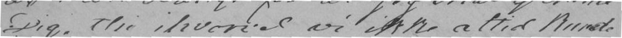Dig. thi ihvorvel vi ikke altid kunde
